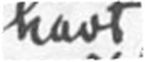havt
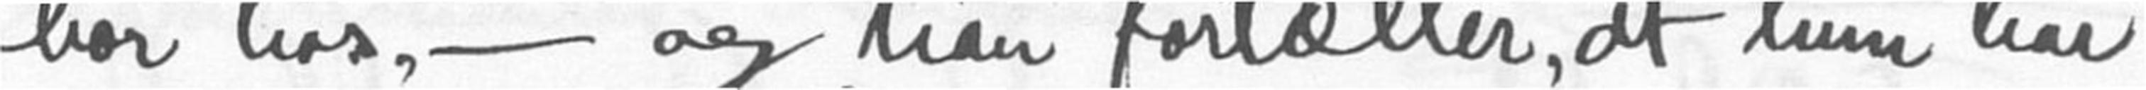bor hos, - og han fortæller, at hun har
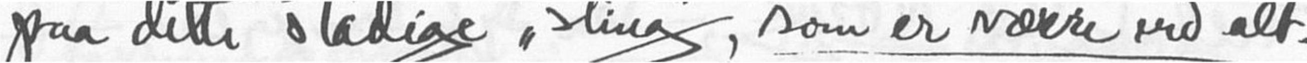paa dette stadige "sting", som er værre end alt.
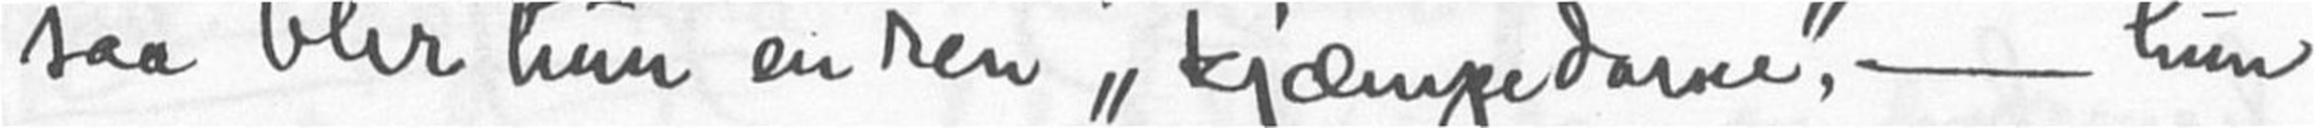saa blir hun en ren "kjæmpedame", - hun
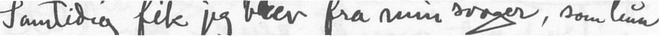Samtidig fik jeg brev fra min svoger, som hun
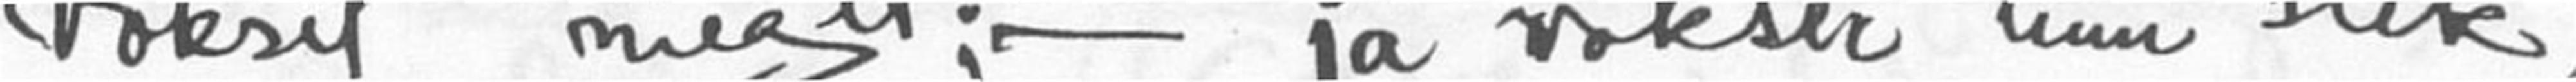vokset meget; - ja vokser hun slik,
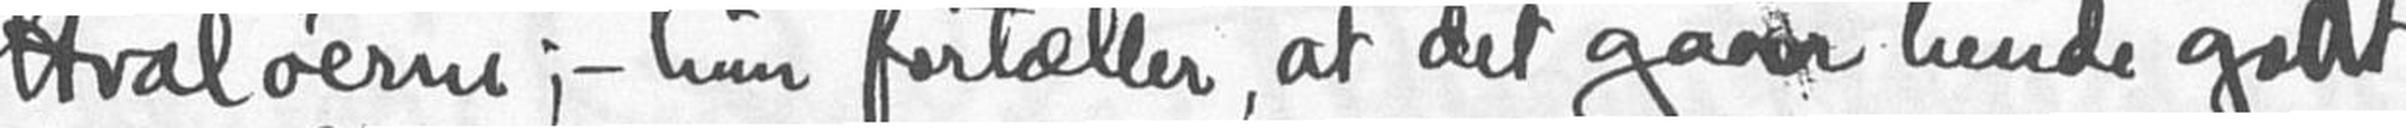Hvaløerne; - hun fortæller, at det gaar hende godt
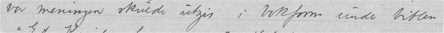var meningen skulde utgis i bokform under titlen
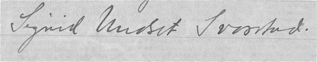Sigrid Undset Svarstad.
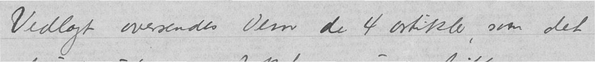Vedlagt oversendes Dem de 4 artikler, som det
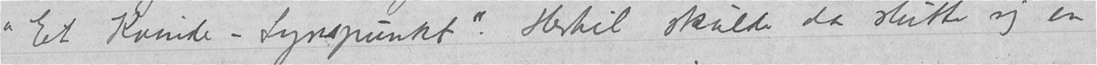"Et Kvinde-Synspunkt". Hertil skulde da slutte sig en
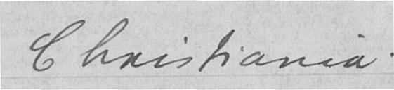Christiania.
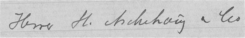Herrer H. Aschehoug & Co
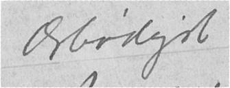Ærbødigst
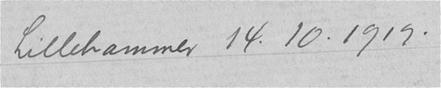Lillehammer 14.10.1919.
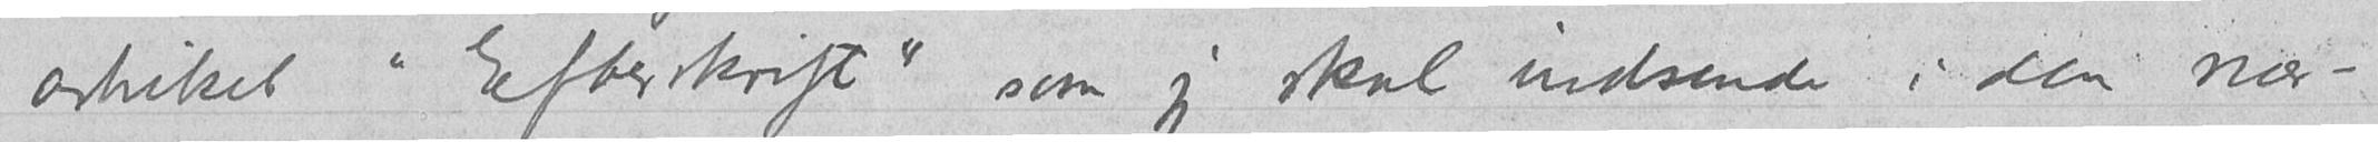artikel "Efterskrift" som jeg skal indsende i den nær–
